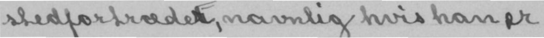stedfortrædetr, navnlig hvis han er
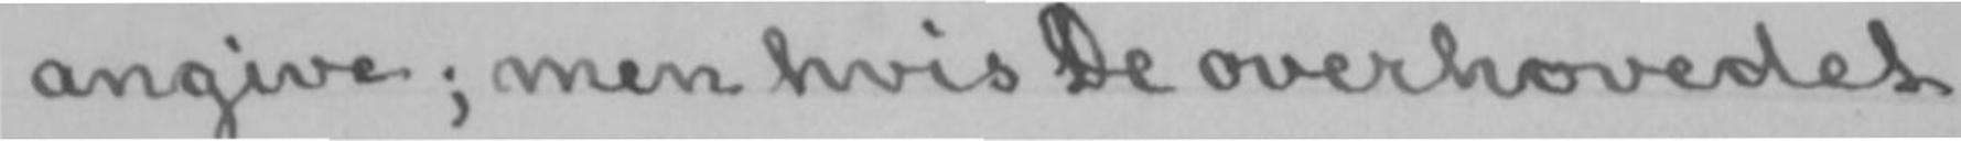angive; men hvis De overhovedet
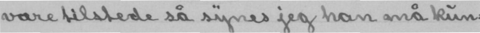være tilstede så synes jeg han må kun-
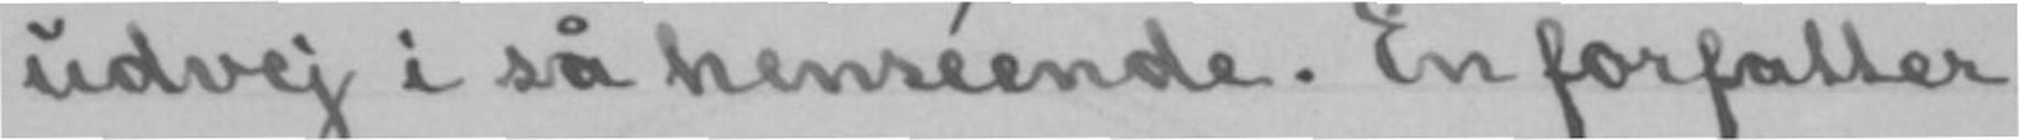udvej i så henséende. En forfatter
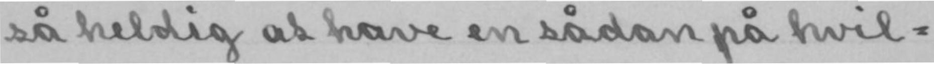så heldig at have en sådan på hvil-
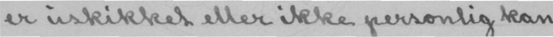er uskikket eller ikke personlig kan
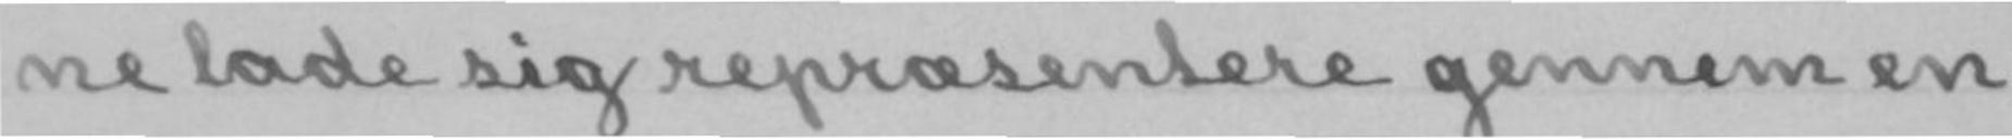ne lade sig repræsentere gennem en
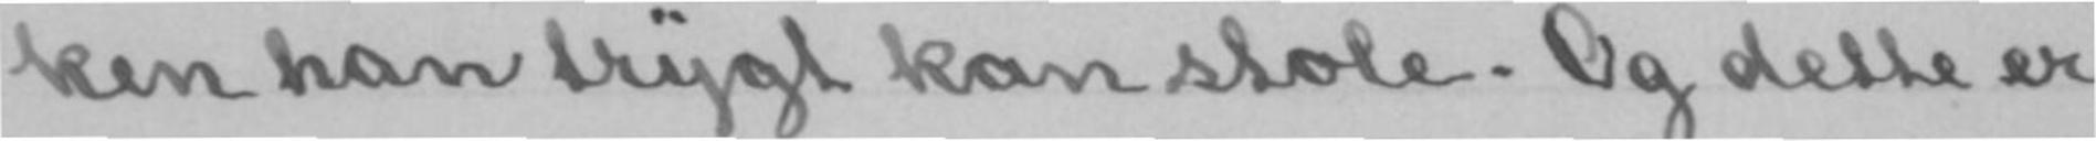ken han trygt kan stole. Og dette er
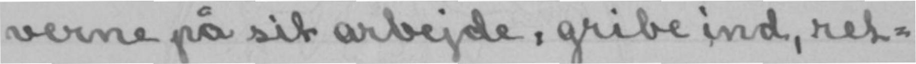verne på sit arbejde, gribe ind, ret-
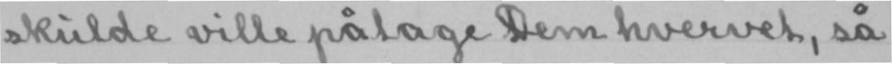skulde ville påtage Dem hvervet, så
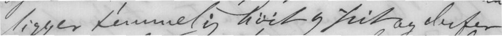ligger temmelig høit og frit og derfor
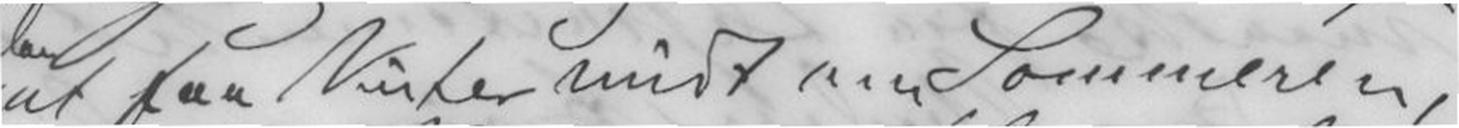at faa Vinter midt om Sommeren,
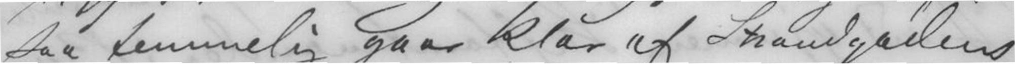saa temmelig gaar klar af Strandgadens
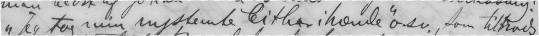Jeg tog min nystemte Cithar ihænde osv., som tiltrods
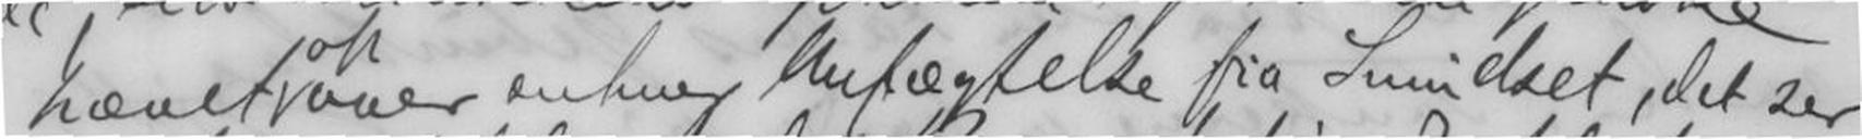hævet op over enhver anfægtelse fra Smudset, det ser
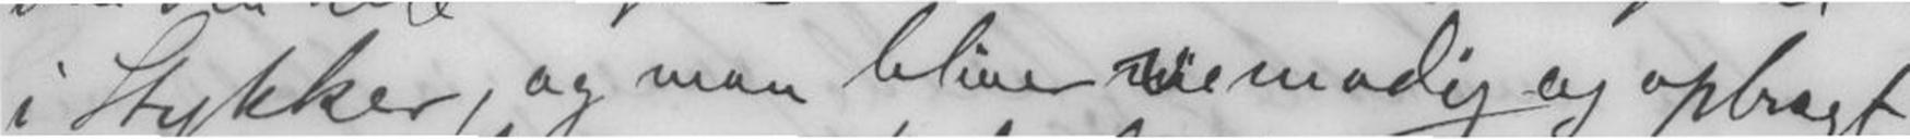i Stykker, og man bliver vemodig og opbragt
